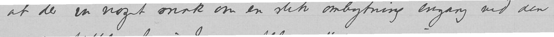at der var noget snak om en slik ombytning engang ved den
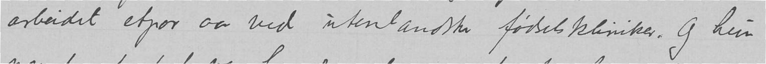arbeidet etpar aar ved utenlandske fødselskliniker. Og hun
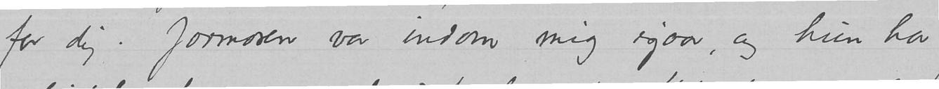for dig. Jormoren var indom mig igaar, og hun har
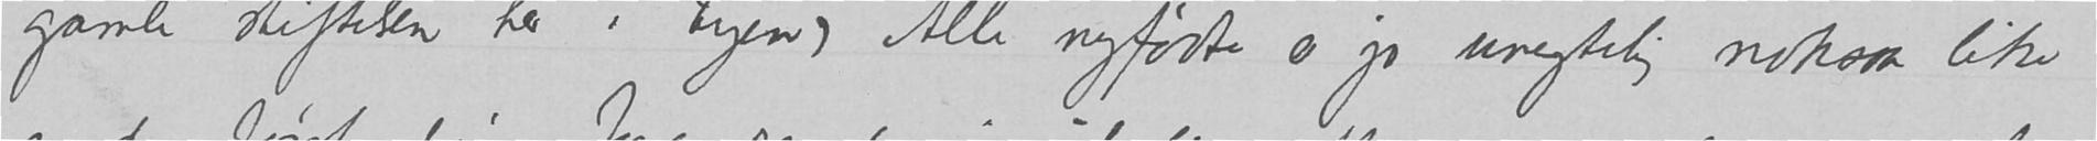gamle stiftelsen her i byen) Alle nyfødte er jo unegtelig noksaa like
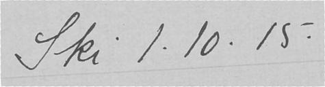Ski 1.10.15.
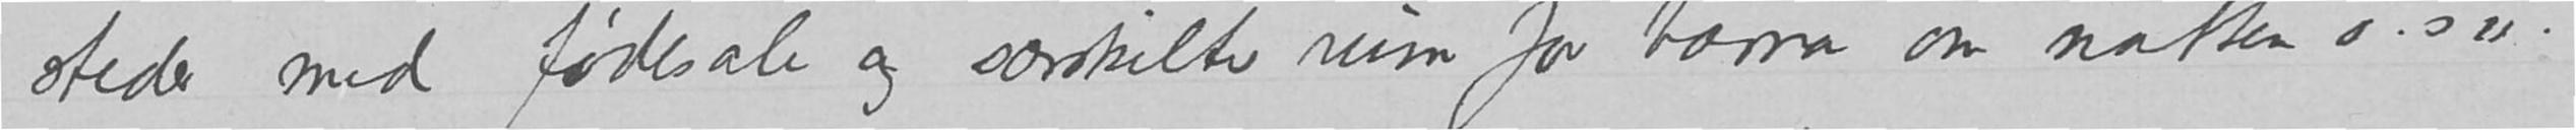steder med fødesale og særskilte rum for barna om natten o.s.v.
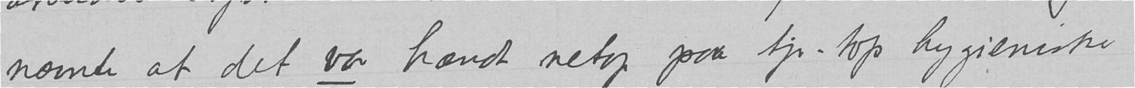nævnte at det var hændt netop paa tip-top hygieniske
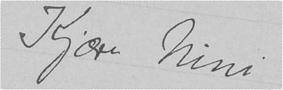Kjære Nini,
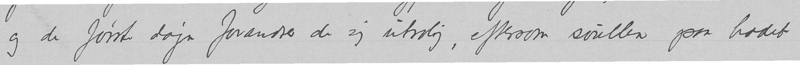og de første døgn forandrer de sig utrolig, eftersom svullen paa hodet
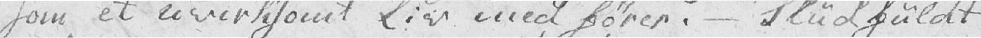som et uvirksomt Liv med fører. – Sludfuldt
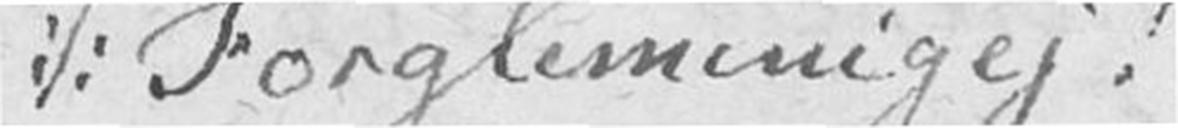:/: Forglemmigej!
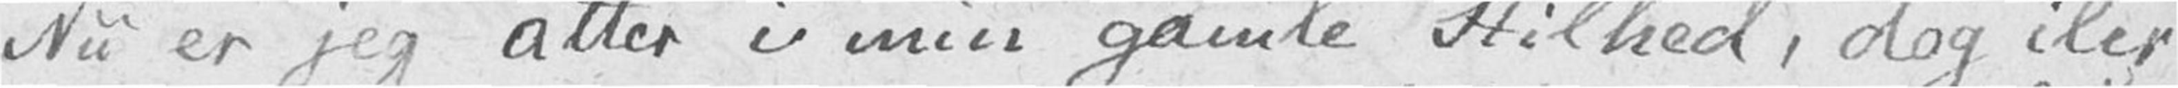Nu er jeg atter i min gamle Stilhed, dog iler
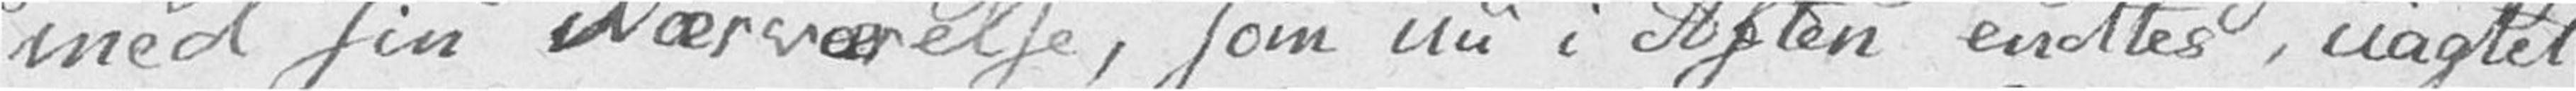med sin Nærværelse, som nu i Aften endtes, uagtet
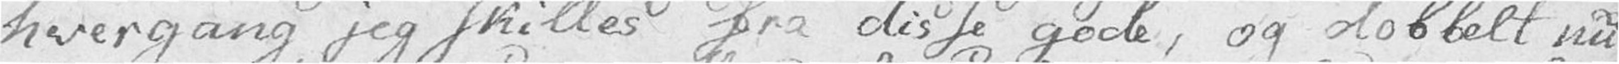hvergang jeg skilles fra disse gode, og dobbelt nu
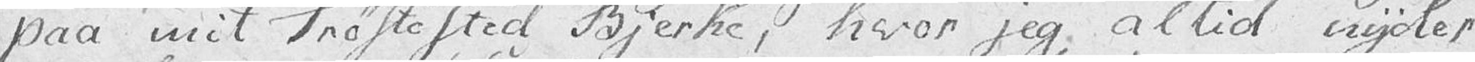paa mit Trøstested Bjerke, hvor jeg altid nyder
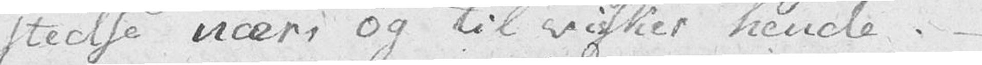stedse nær, og tilvisker hende. –
